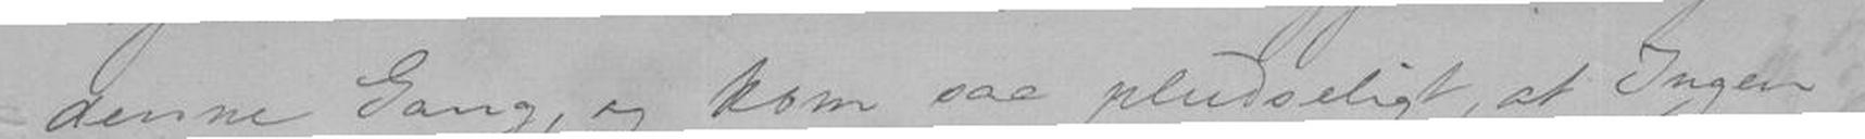denne Gang, og kom saa pludseligt, at Ingen
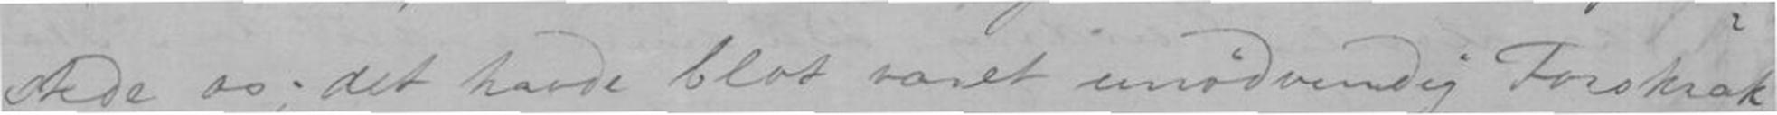stede os; det havde blot været unødvendig Forskræk
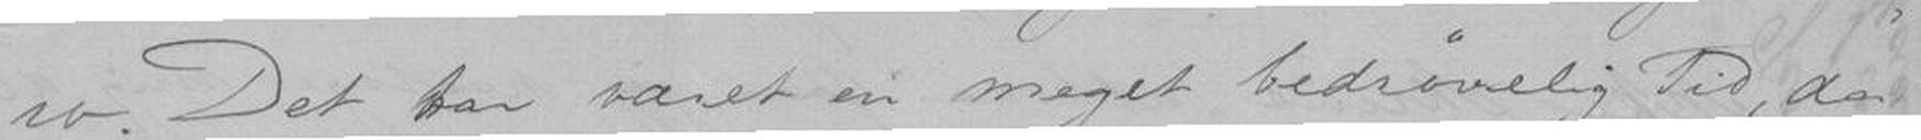ro. Det har været en meget bedrøvelig Tid, da
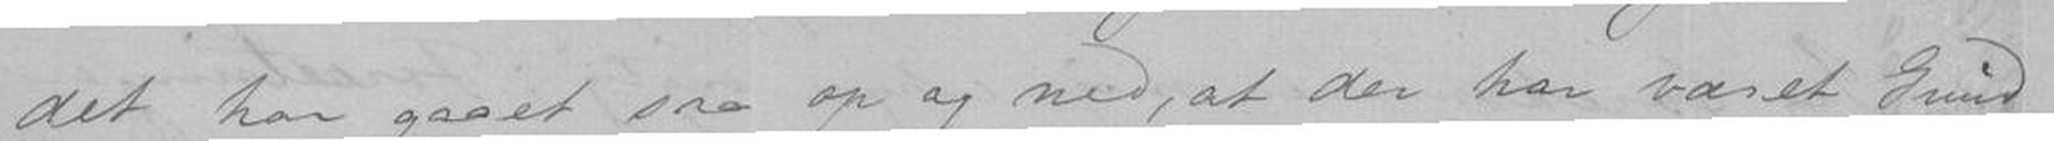det har gaaet saa op og ned, at der har været Grund
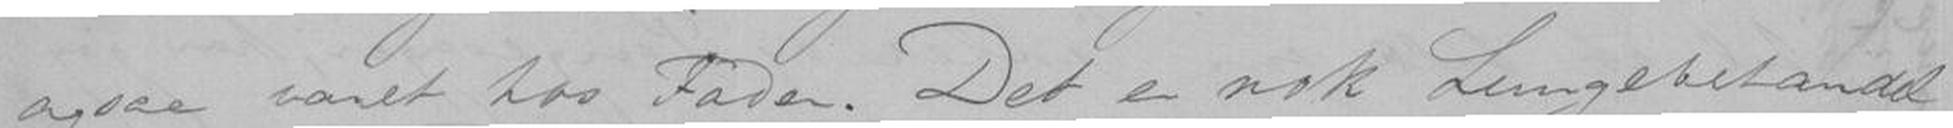ogsaa været hos Fader. Det er nok Lungebetændel-
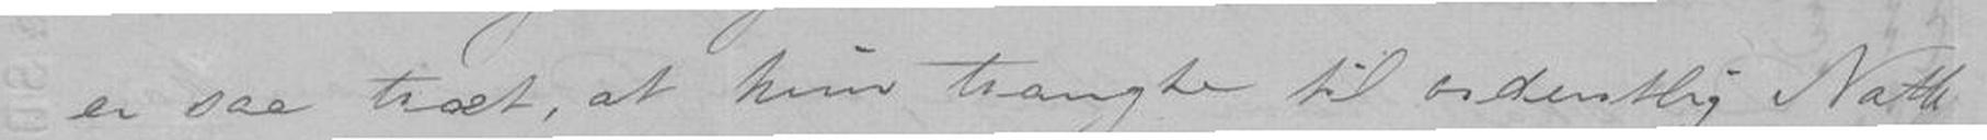er saa træt, at hun trængte til ordentlig Natte-
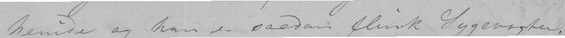herude og han er saadan flink Sygevogter;
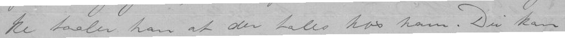ke taaler han at der tales hos ham; Du kan
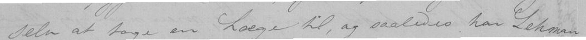selv at tage en Læge til, og saaledes har Lehman
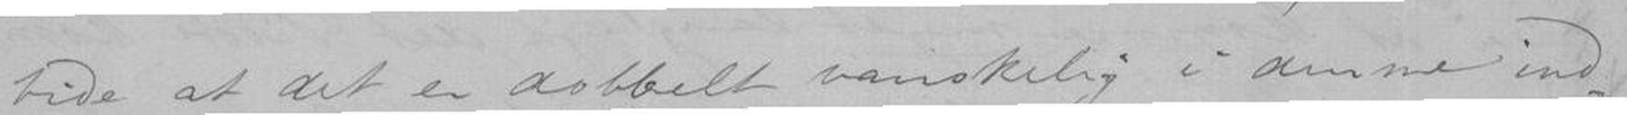tide at det er dobbelt vanskelig i denne ind-
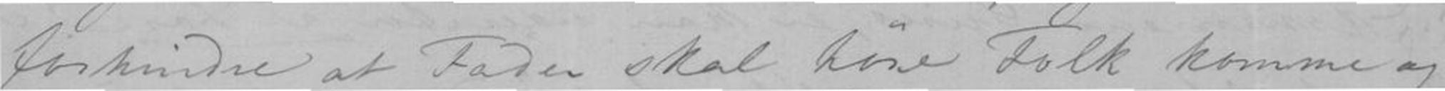forhindre at Fader skal høre Folk komme og
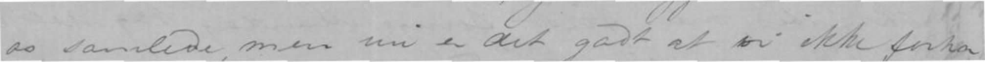os samlede, men nu er det godt at vi ikke forha-
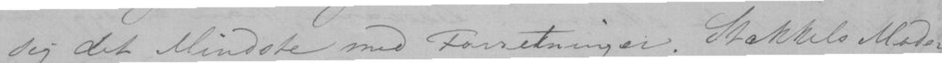sig det Mindste med Forretninger. Stakkels Moder
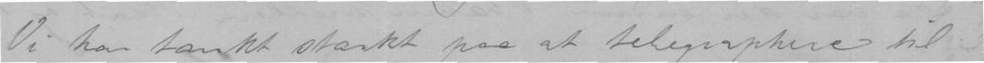Vi har tænkt stærkt paa at telegraphere til
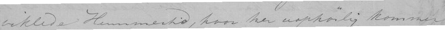viklede Hummertid, hvor her uophørlig kommer
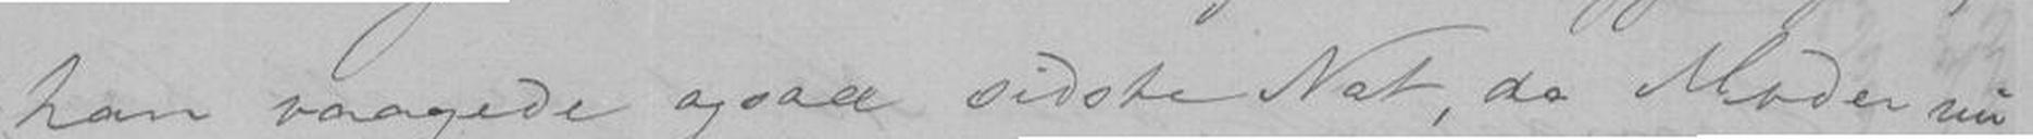han vaagede ogsaa sidste Nat, da Moder nu
